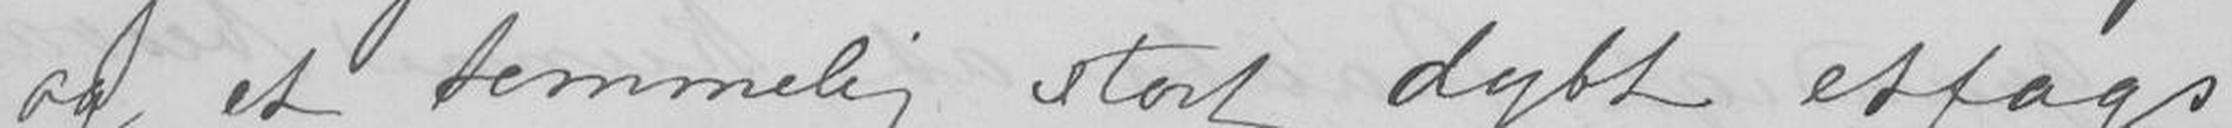og et temmeligg stort dypt etfags
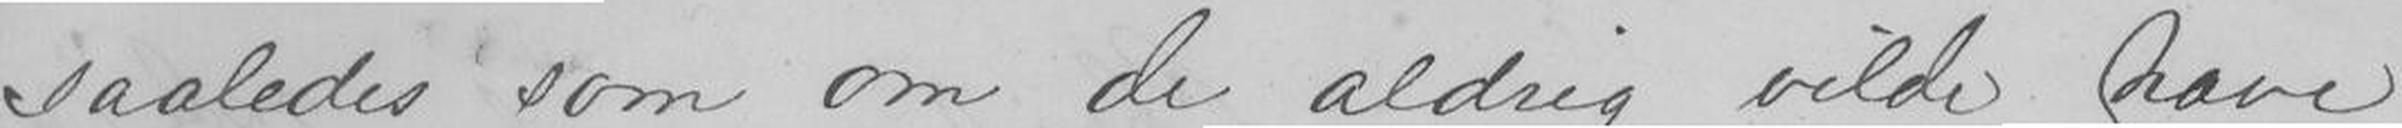saaledes, som om de aldrig vilde have
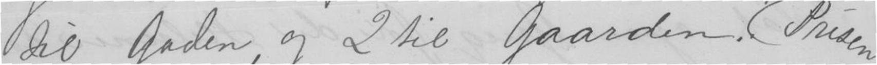til Gade, og 2 til Gaarden. Prisen
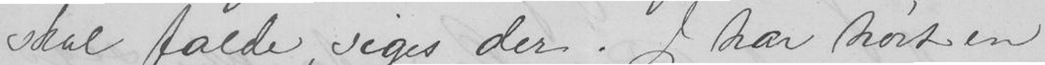skal falde, siges der. Jeg har hørt en
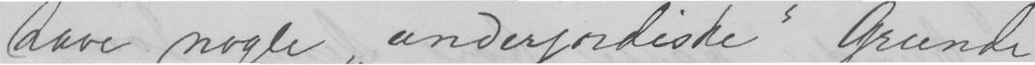have nogle underjordiske Grunde,
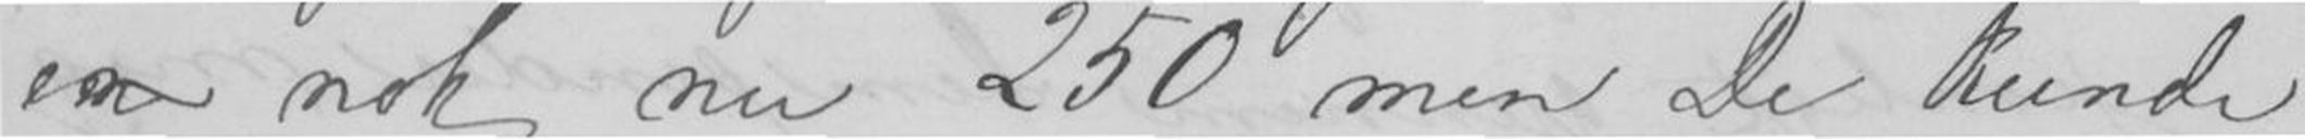er nok nu 250 men De kunde
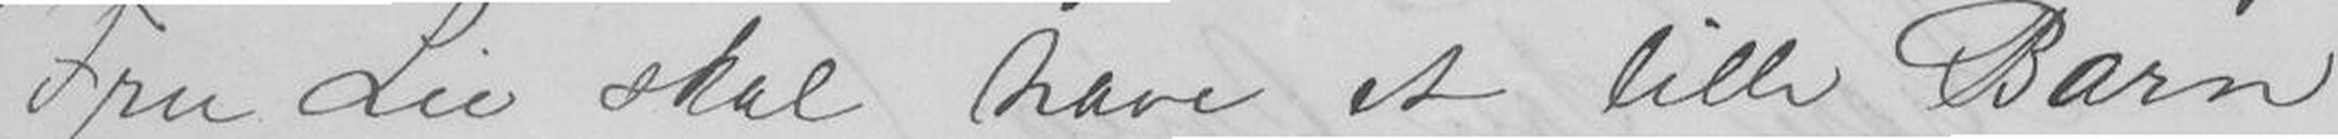Fru Lie skal have et lille Barn
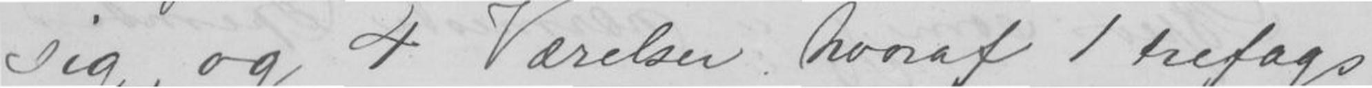sig, og 4 Værelser hvoraf 1 trefags
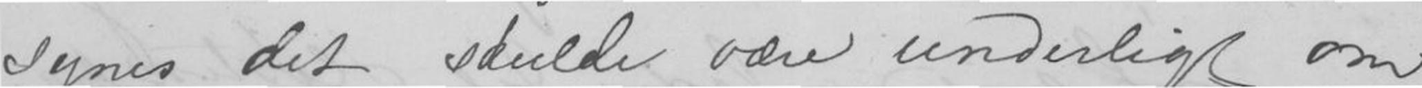synes det skulde være underligt om
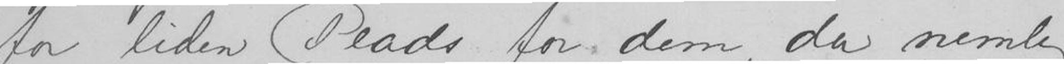for liden Plads for dem, da nemlig
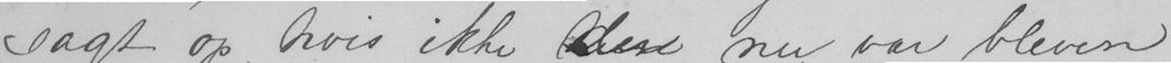sagt op, hvis ikke den nu var bleven
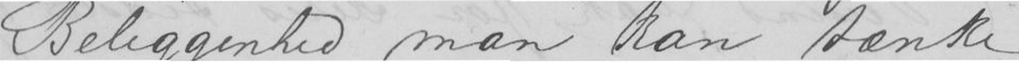Beliggenhed man kan tænke
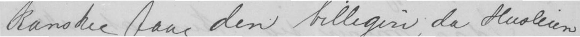kanske faa den billigere, da Husleien
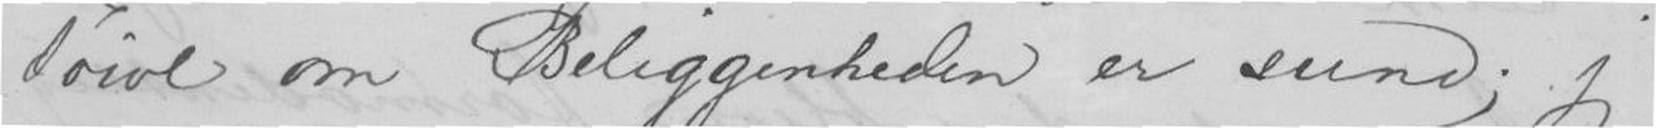Tvivl om Beliggenheden er sund; jeg
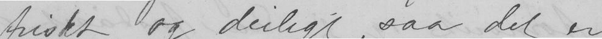friskt og deiligt, saa det er
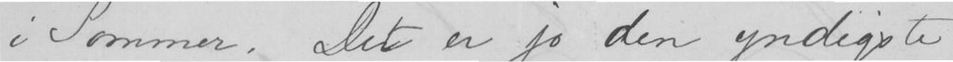i Sommer. Det er jo den yndigste
